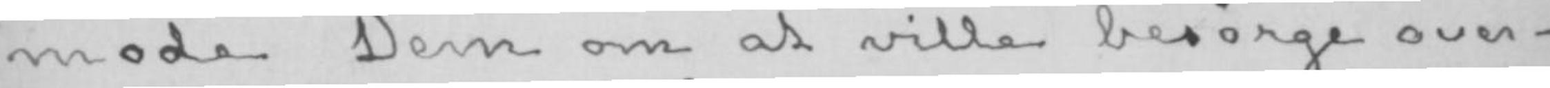mode Dem om at ville besørge over-
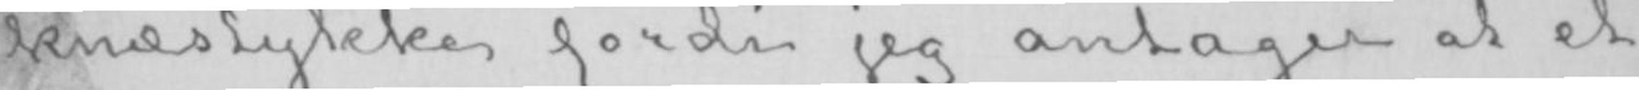knæstykke fordi jeg antager at et
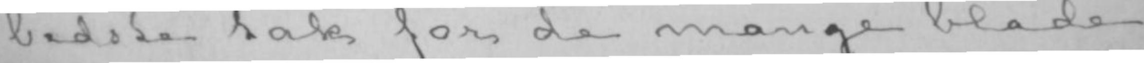bedste tak for de mange blade,
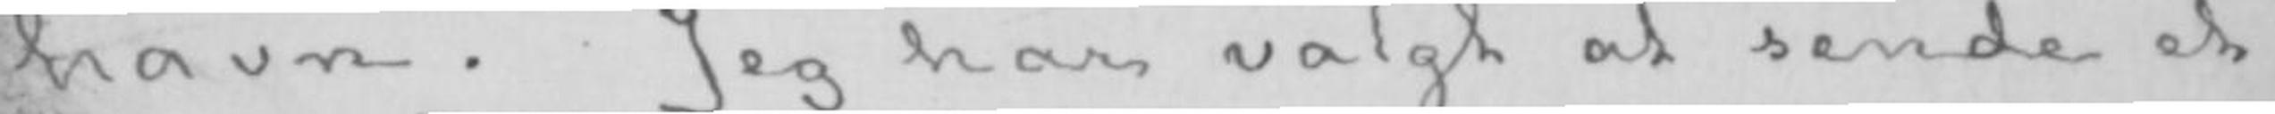havn. Jeg har valgt at sende et
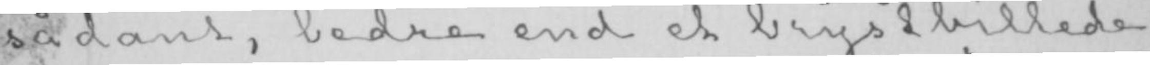sådant, bedre end et brystbillede,
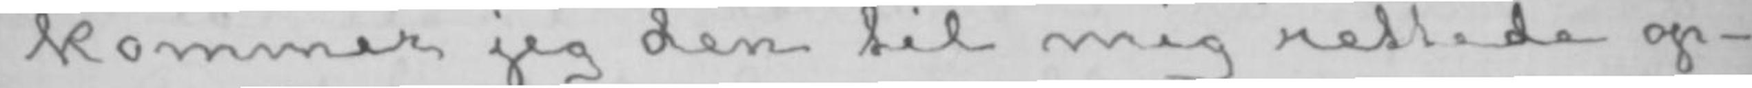kommer jeg den til mig rettede op-
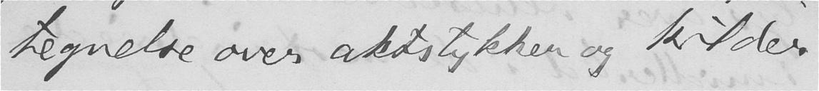tegnelse over aktstykker og kilder
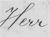Herr
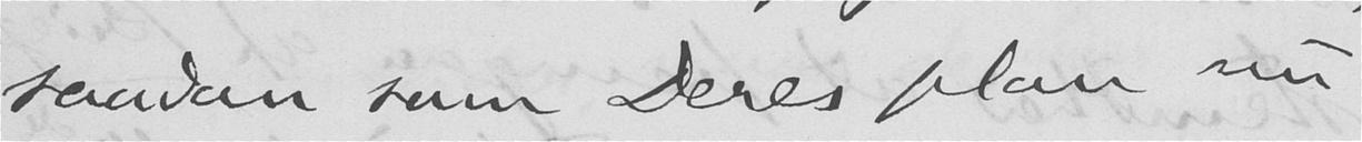saadan som Deres plan nu
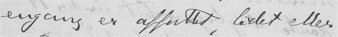engang er affattet, lidet eller
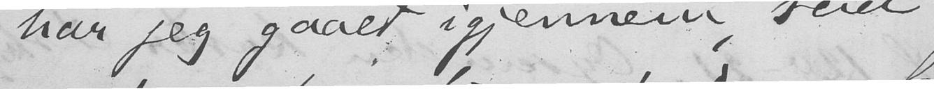har jeg gaaet igjennem, saa
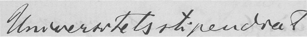Universitetsstipendiat
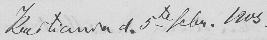Kristiania d. 5te febr. 1905.
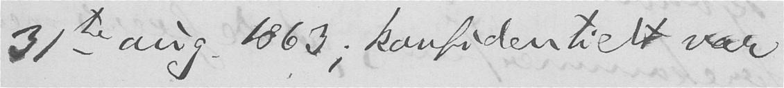31te aug. 1863; konfidentielt var
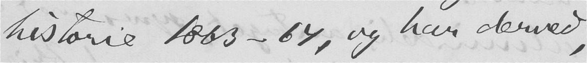historie 1863-64, og har derved,
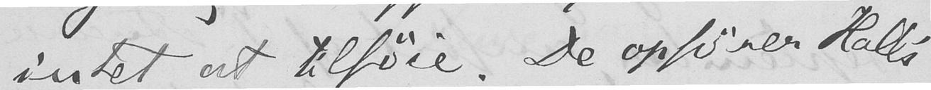intet at tilføie. De opfører Hall's
